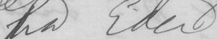fra Eders
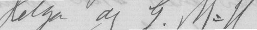Helga og G. M-H
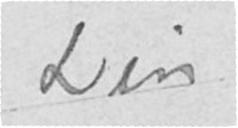Din
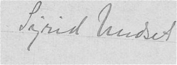Sigrid Undset.
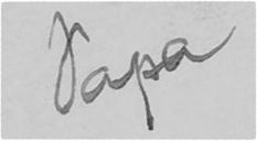Papa
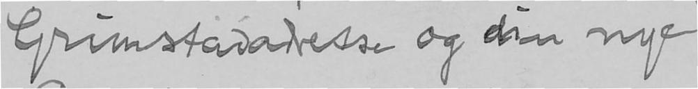Grimstadadresse og din nye
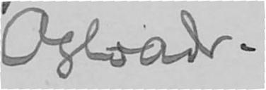Osloadr.
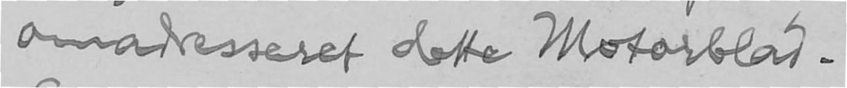omadresseret dette Motorblad.
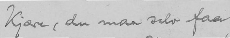Kjære, du maa selv faa
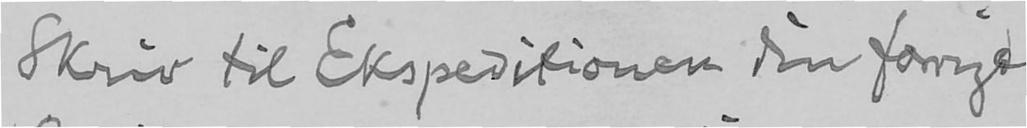Skriv til Ekspeditionen din forrige
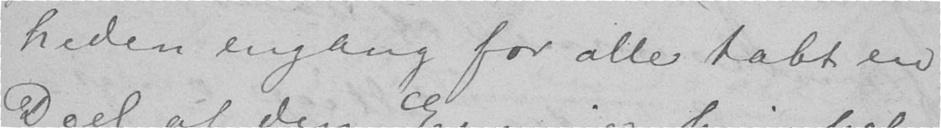heden engang for alle tabt en
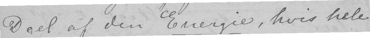Deel af den Energie, hvis hele
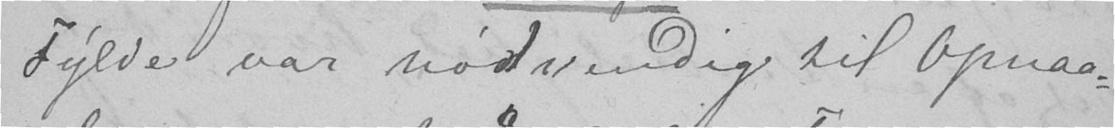Fylde var nødvendig til Opnaa-
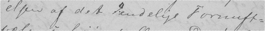elsen af det endelige Fornuft-
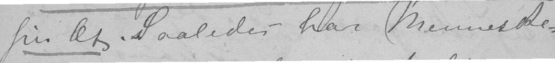sin Æt. Saaledes har Menneske-
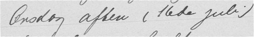Onsdag aftese (16de juli)
